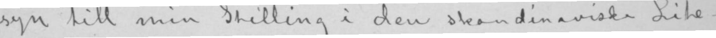syn till min Stilling i den skandinaviske Lite-
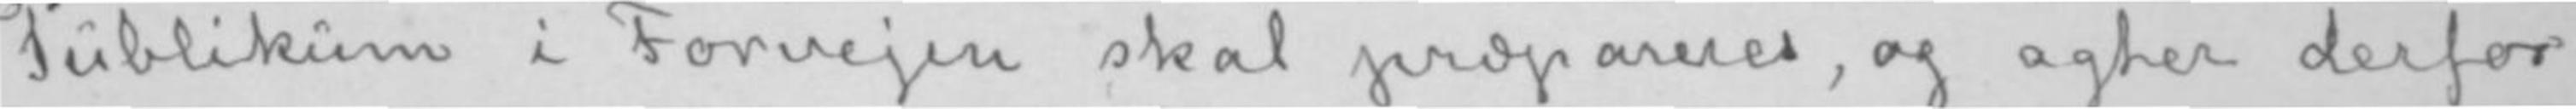Publikum i Forvejen skal præpareres, og agter derfor
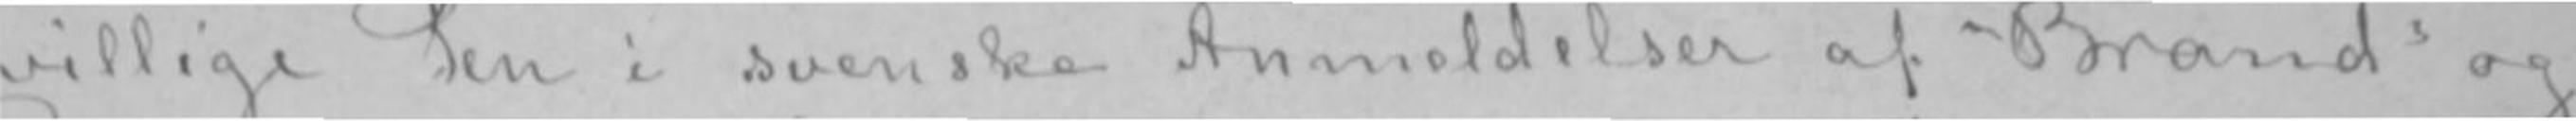villige Pen i svenske Anmeldelser af «Brand» og
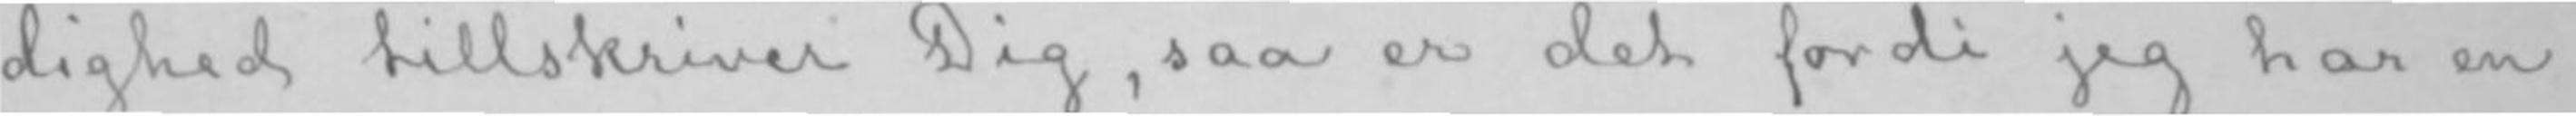dighed tillskriver Dig, saa er det fordi jeg har en
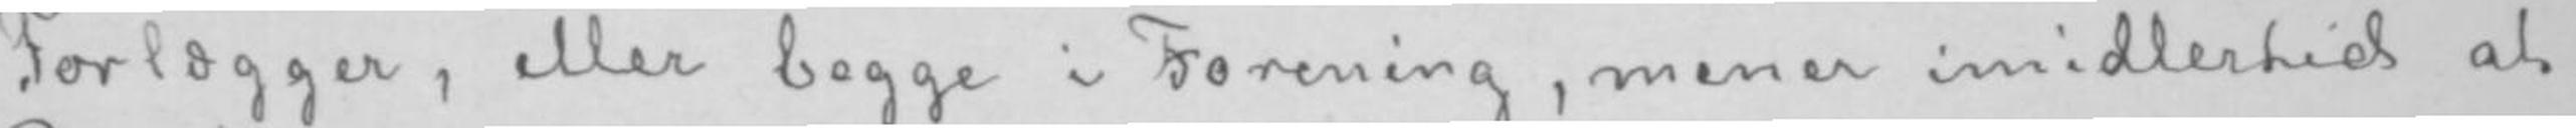Forlægger, eller begge i Forening, mener imidlertid at
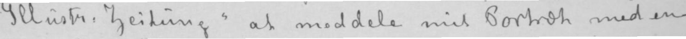«Illustr: Zeitung» at meddele mit Portræt med en
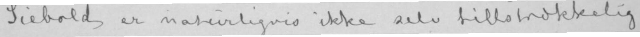Siebold er naturligvis ikke selv tillstrækkelig
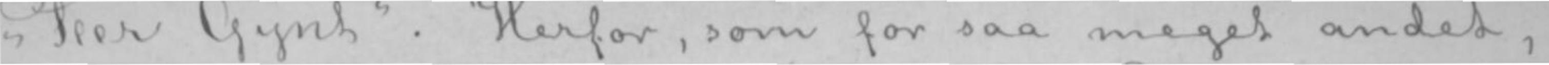«Peer Gynt». Herfor, som for saa meget andet,
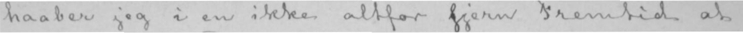haaber jeg i en ikke altfor fjern Fremtid at
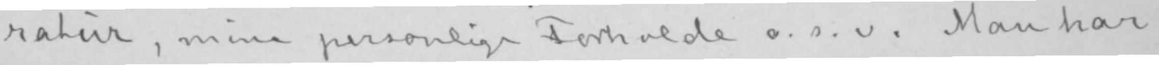ratur, mine personlige Forholde o. s. v. Man har
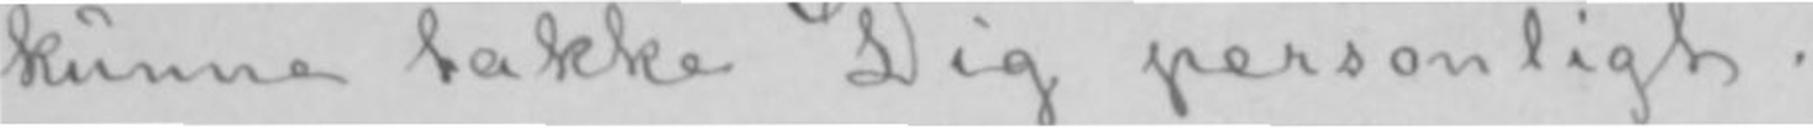kunne takke Dig personligt.
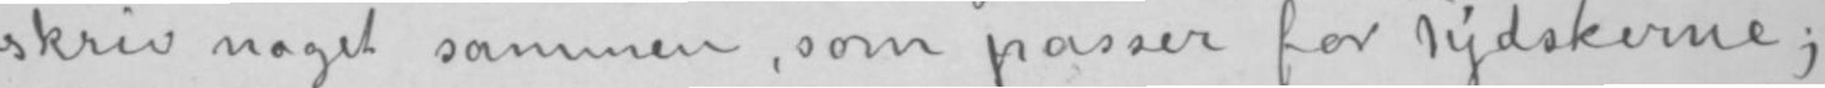skriv noget sammen, som passer for Tydskerne;
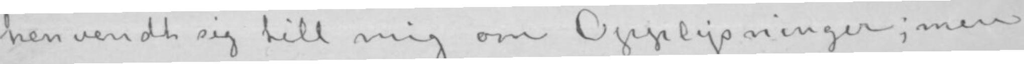henvendt sig till mig om Opplysninger; men
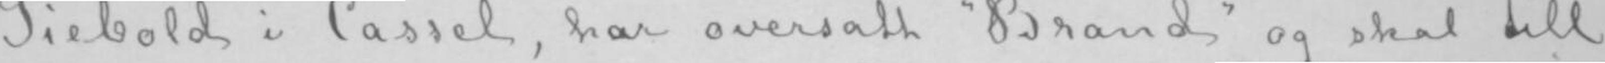Siebold i Cassel, har oversatt «Brand» og skal till
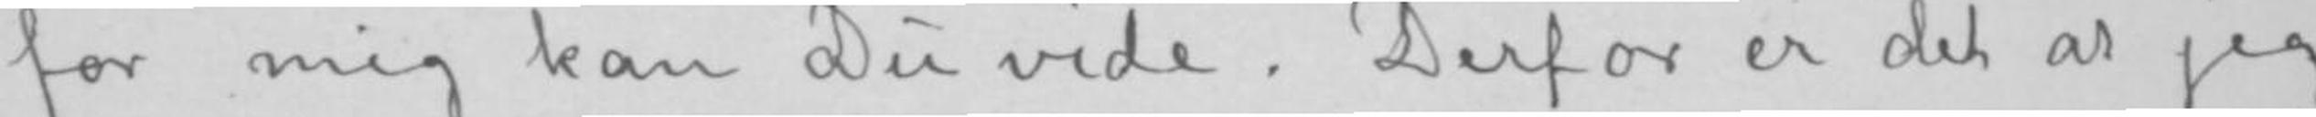for mig kan Du vide. Derfor er det at jeg
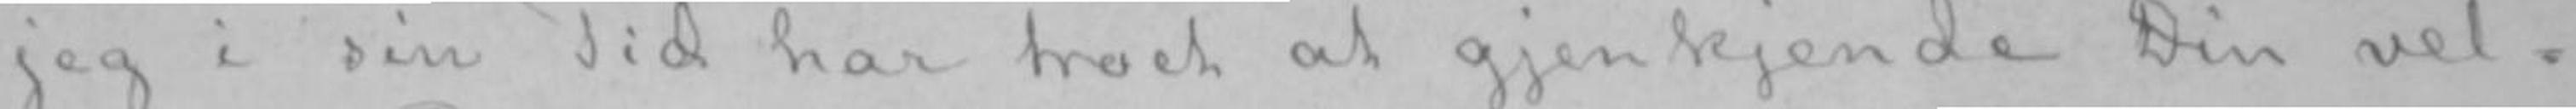jeg i sin Tid har troet at gjenkjende Din vel-
